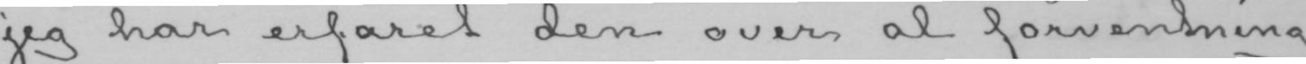jeg har erfaret den over al forventning
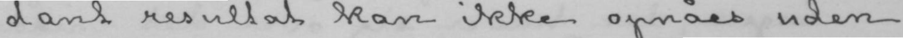dant resultat kan ikke opnåes uden
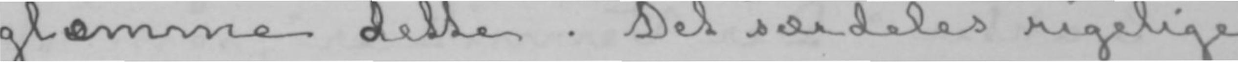glemme dette. Det særdeles rigelige
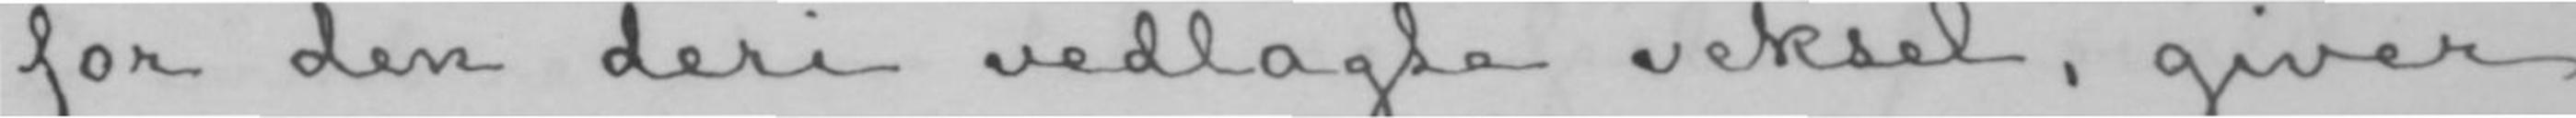for den deri vedlagte veksel, giver
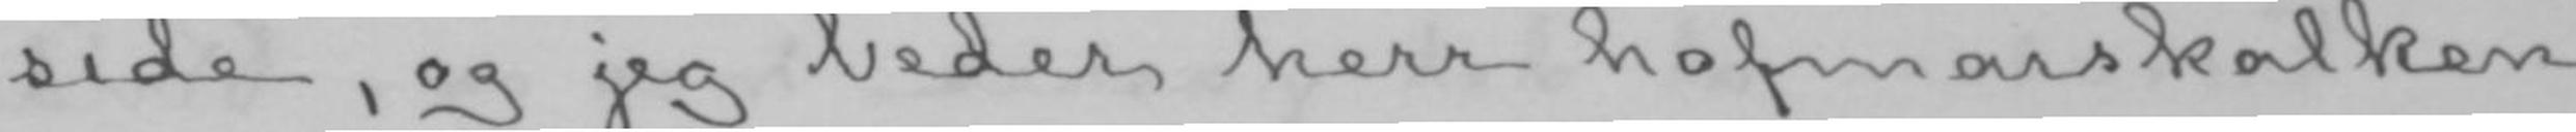side, og jeg beder herr hofmarskalken
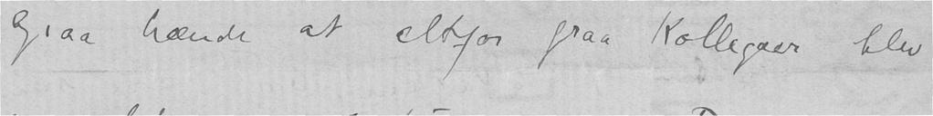ogsaa hænde at altfor graa Kollegaer blev
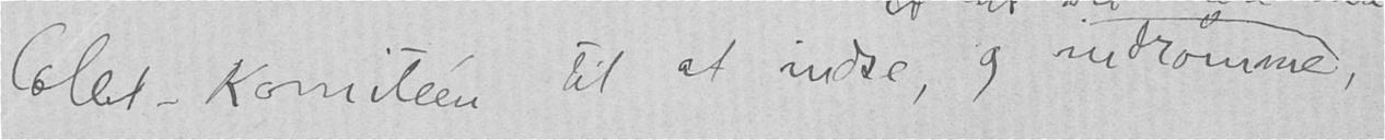Collet-Komitéen til at indse, og indrømme,
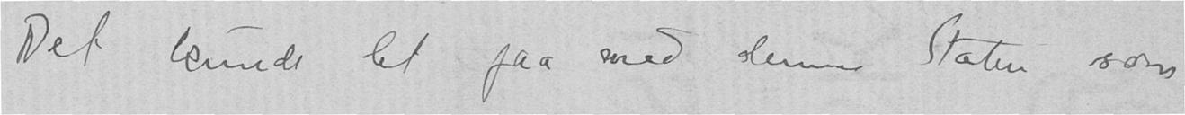Det kunde let gaa med denne Statue som
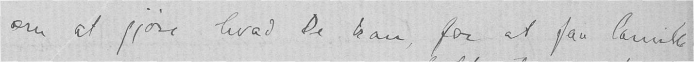om at gjøre hvad De kan, for at faa Camila
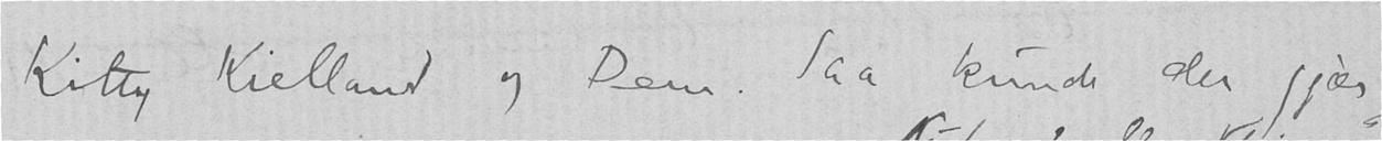Kitty Kielland og Dem. Saa kunde der gjær-
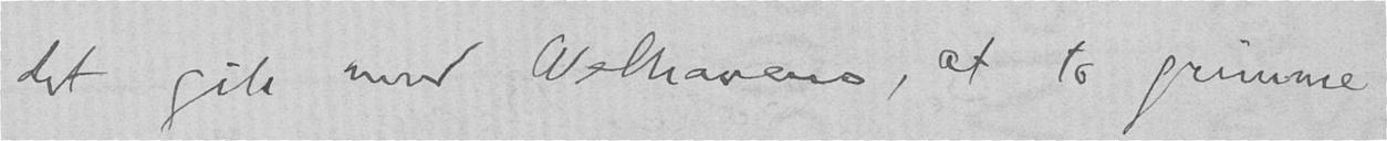det gik med Welhavens, at to grimme
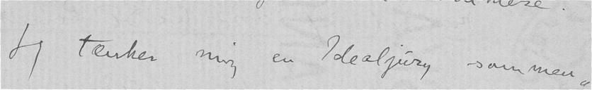Jeg tænker mig en Idealjury sammen-
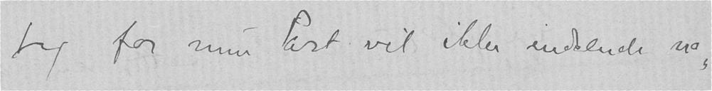Jeg for min Part vil ikke indsende no-
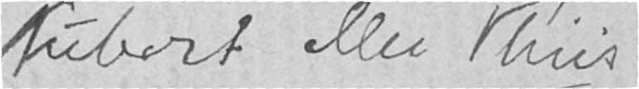Aubert eller Thiis,
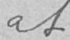at
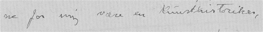ne for mig være en Kunsthistoriker,
